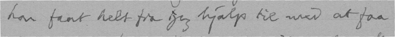har faat helt fra jeg hjalp til med at faa
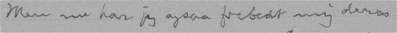Men nu har jeg ogsaa frabedt mig deres
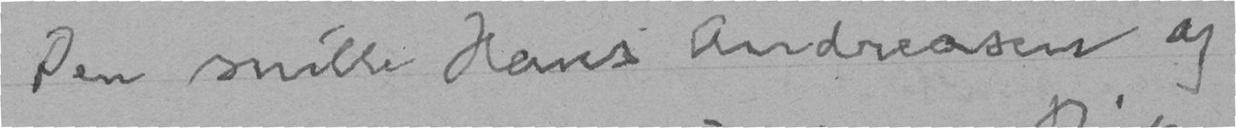Den snille Hans Andreasen og
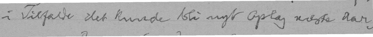i Tilfælde det kunde bli nyt Oplag næste Aar.
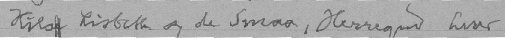Hilse Lisbeth og de Smaa, Herregud hvor
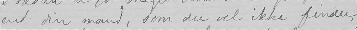end din mand, som du vel ikke finder,
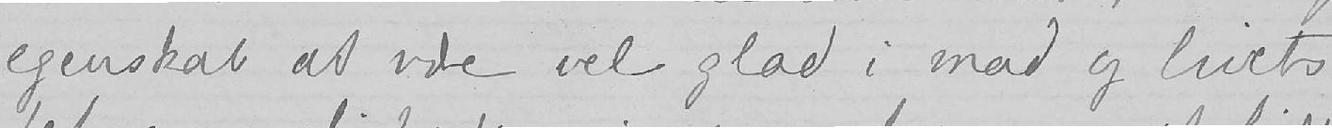egenskab at være vel glad i mad og livets
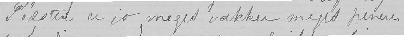Præsten er jo meget vakker meget penere
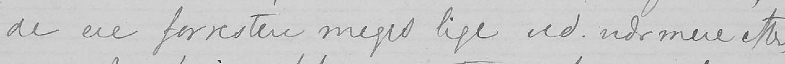de ere forresten meget lige ved nærmere efter-
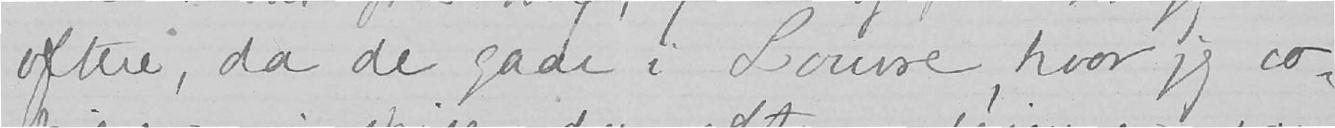oftere, da de gaae i Louvre, hvor jeg co-
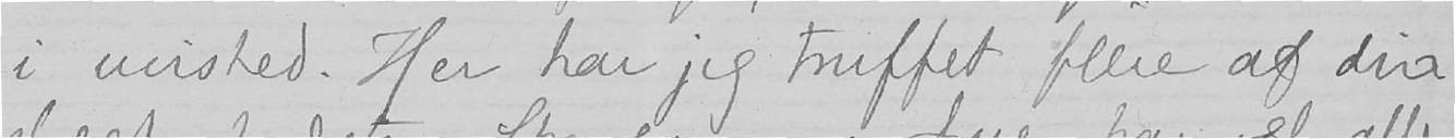i uvished. Her har jeg truffet flere af din
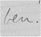ben.
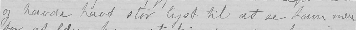og havde havt stor lyst til at se ham mere
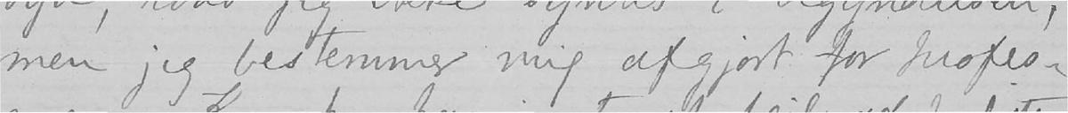men jeg bestemmer mig afgjort for profes-
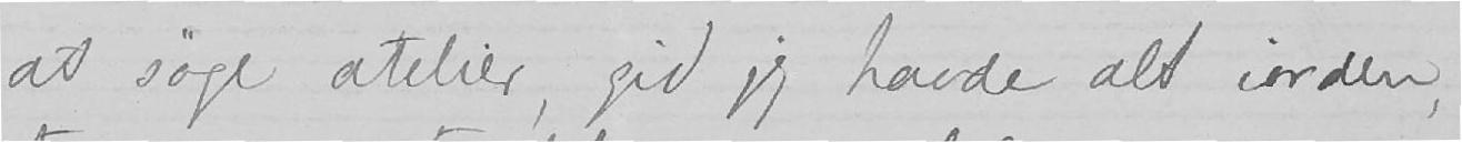at søge atelier, gid jeg havde alt iorden,
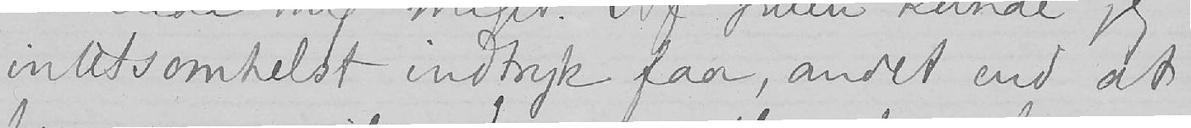intetsomhelst indtryk faa, andet end at
